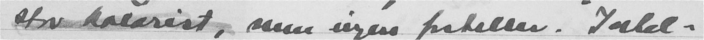stor kolorist, men ingen forteller. Intel-
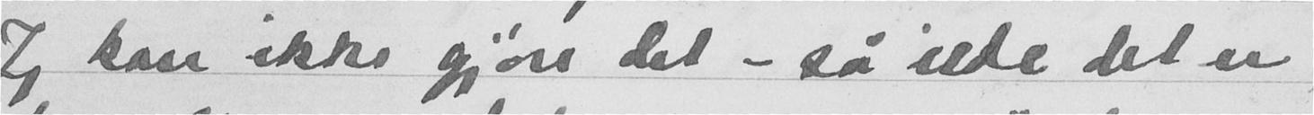Jeg kan ikke gjøre det - så ilde det er
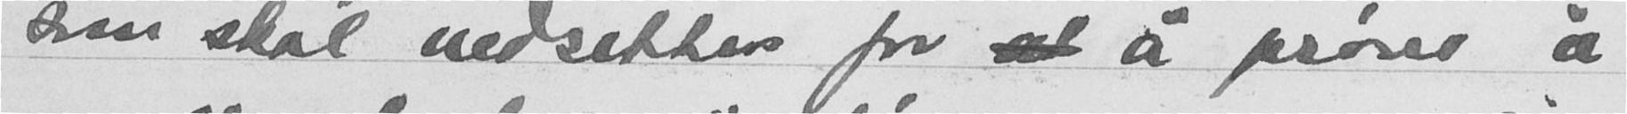som skal nedsettes for å prøve å
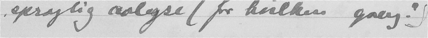sproglig avlegse (for hvilken gang?)
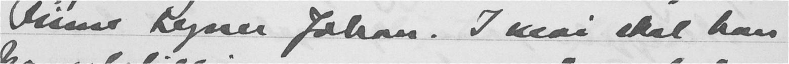Finne tegner Johan. I mai skal han
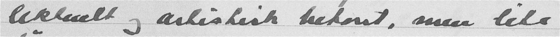lektuelt og artistisk betont, men lite
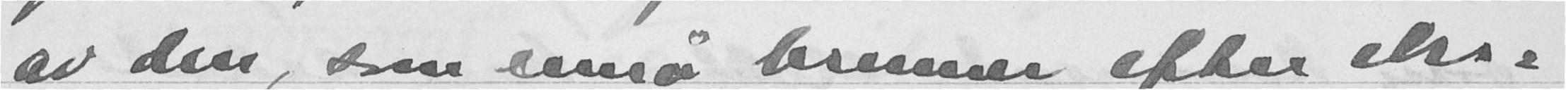av den, som ennå brenner efter eks-
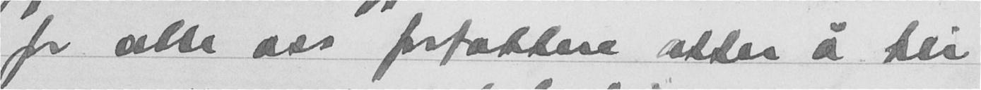for alle oss fortattere atter å bli
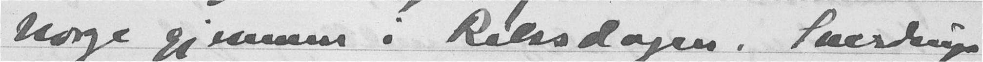Norge gjennem i Riksdagen. Sverdrups
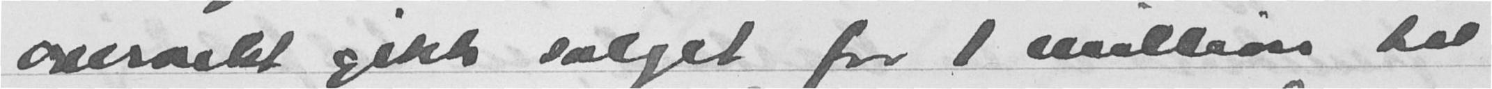overvekt gikk salget for 1 million til
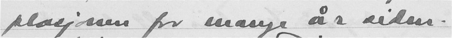plosjonen for mange år siden.
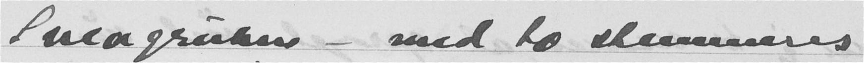Sveagruben - med to stemmers
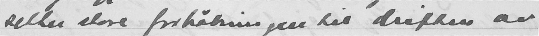setter store forhåpninger til driften av
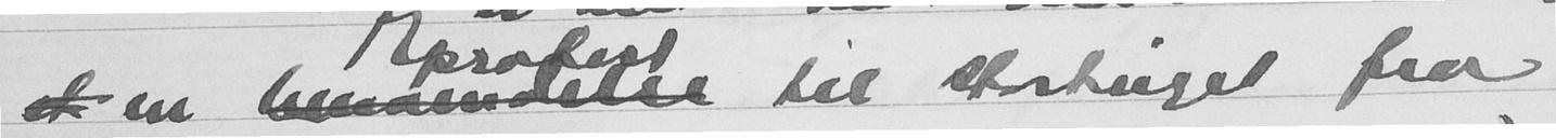en  protest til stortinget fra
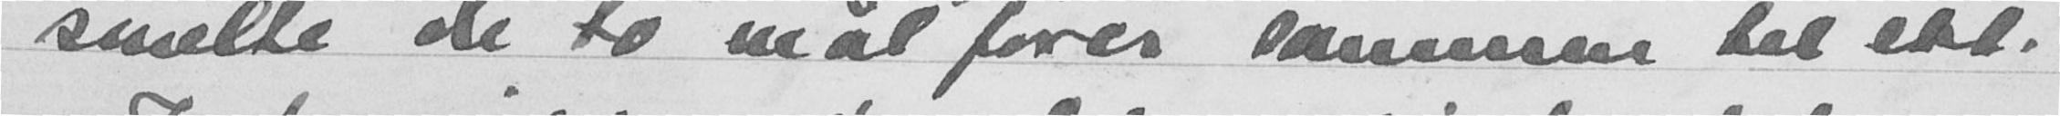smelte de to målførene sammen til ett.
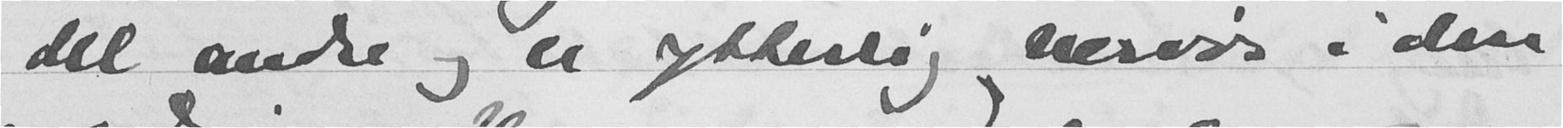del andre og er ytterlig nervøs i den
